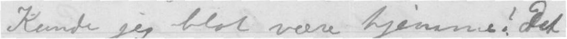Kunde jeg blot være hjemme! Det
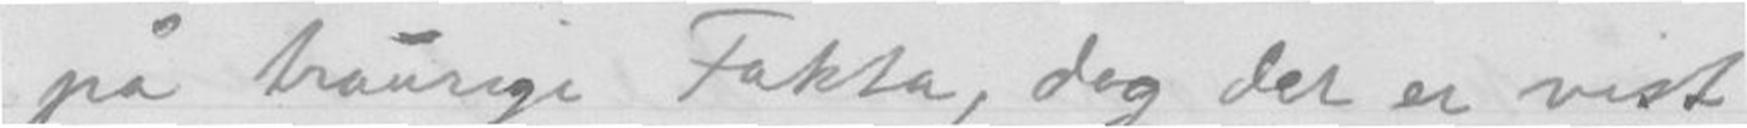på traurige Fakta, dog det er vist
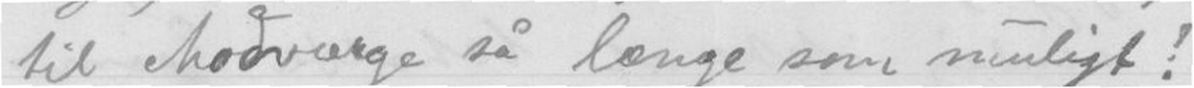til Modværge så længe som muligt!
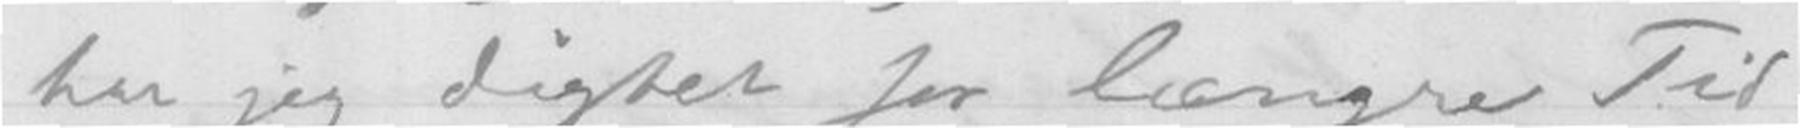har jeg digtet for længre Tid
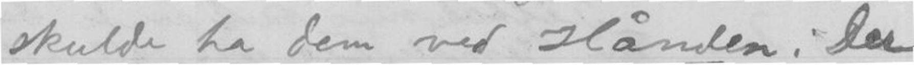skulde ha Dem ved Hånden. Der
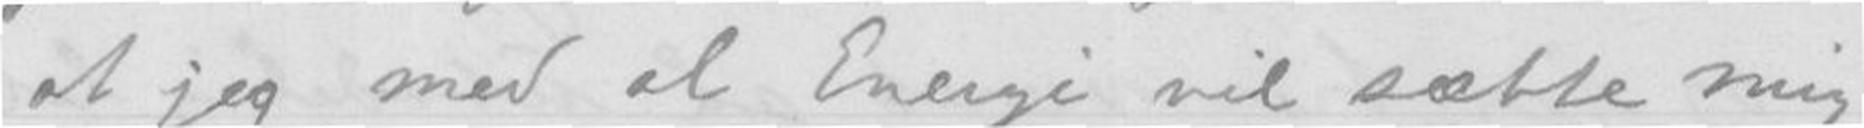at jeg med al Energi vil sætte mig
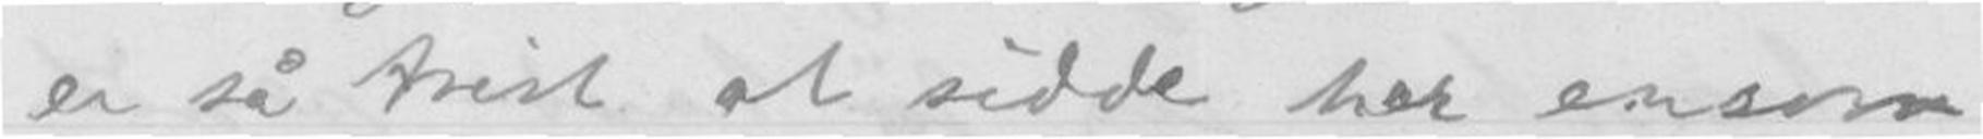er så trist at sidde her ensom
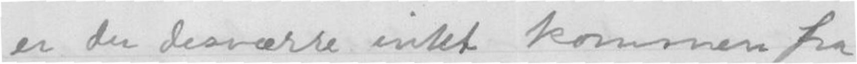er der desværre intet kommen fra
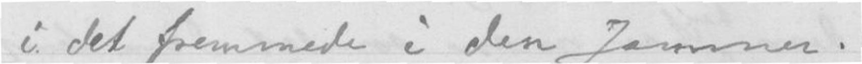i det fremmede i den Jammer.
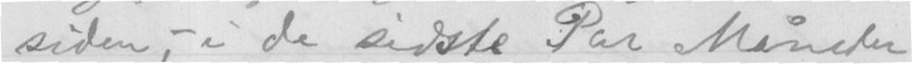siden, - i de sidste Par Måneder
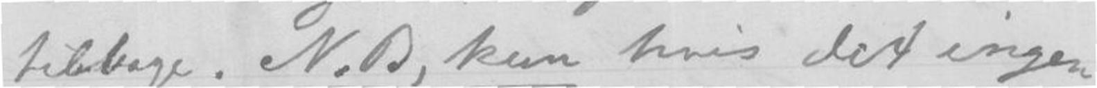tilbage. N.B, kun hvis det ingen
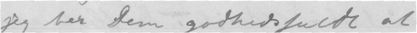jeg ber Dem godhedsfuldt at
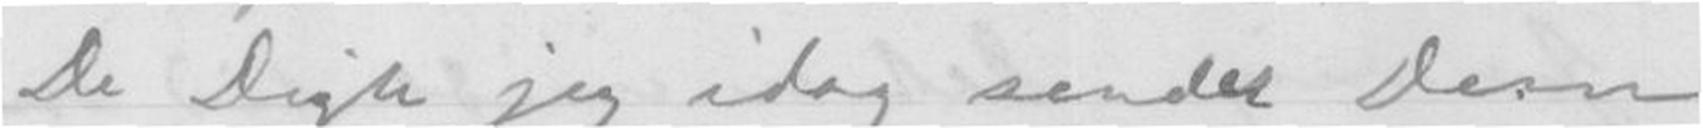De Digte jeg idag sender Dem
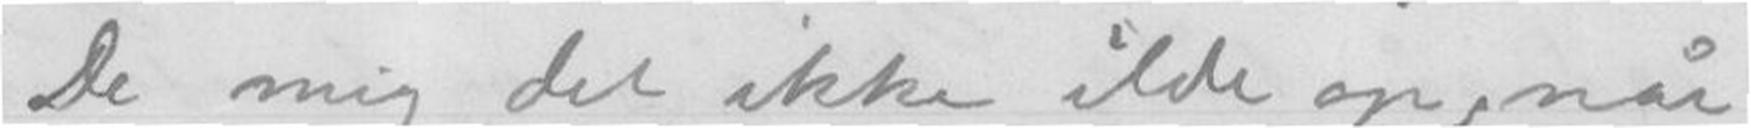De mig det ilde op, når
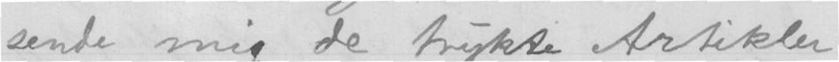sende mig de trykte Artikler
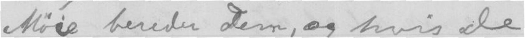Møie bereder Dem, og hvis De
